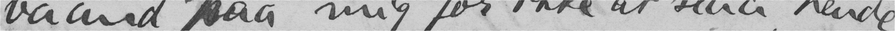baand paa mig for ikke at slaa hende
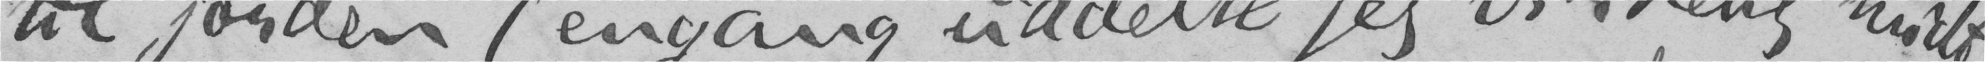til jorden (engang uddelte jeg virkelig midt
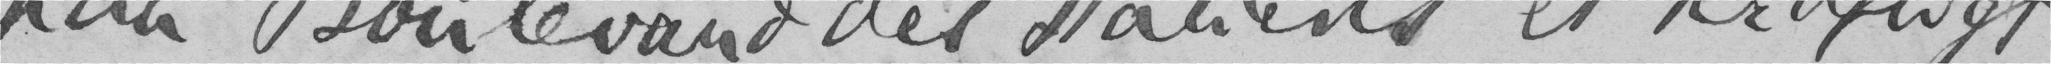paa Boulevard des Italiens et kraftigt
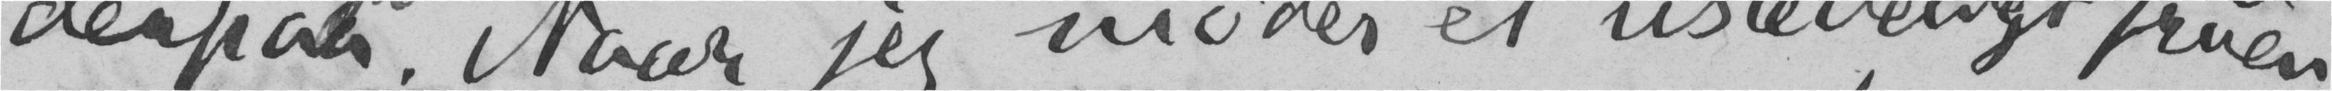derpaa. Når jeg møder et usædeligt fruen-
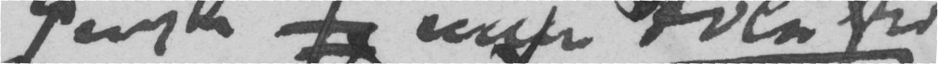ganske min Følelse
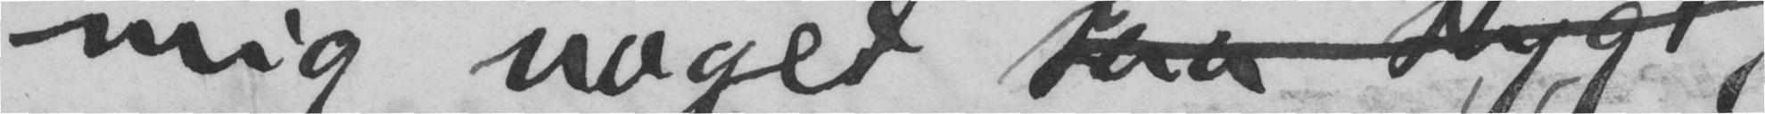mig noget saa stygt,
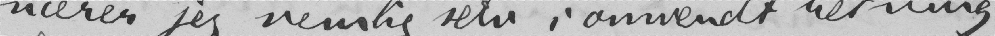nærer jeg nemlig selv i omvendt retning
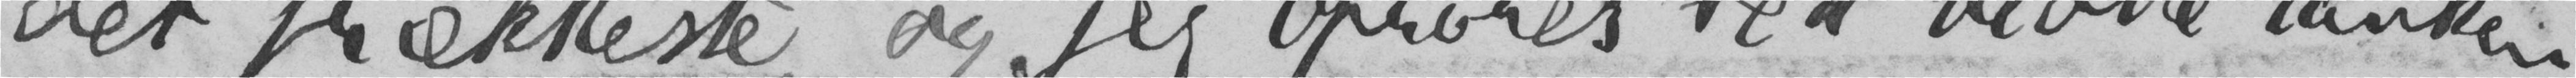det frækkeste, og jeg oprøres ved blotte tanken
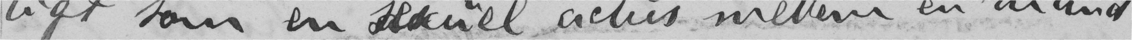ligt som en sexuel actus mellem en mand
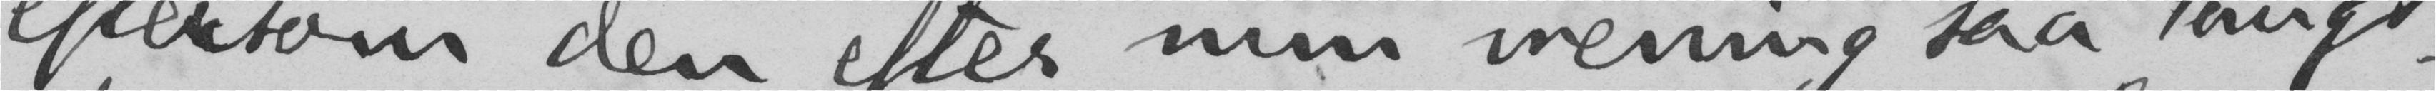eftersom den efter min mening saa langt-
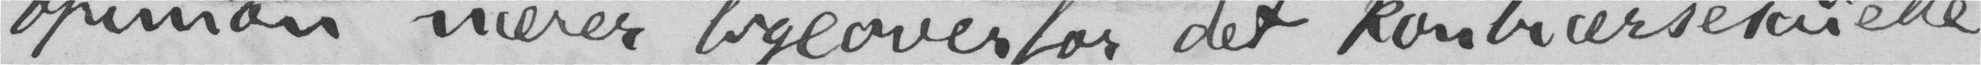opinion nærer ligeoverfor det kontrærsexuelle
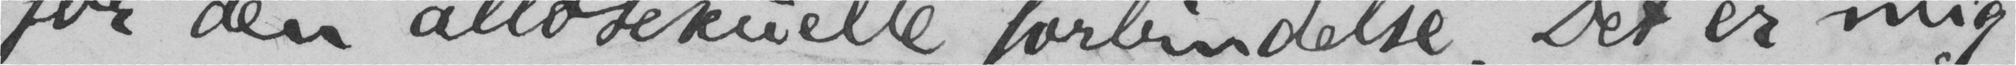for den allosexuelle forbindelse. Det er mig
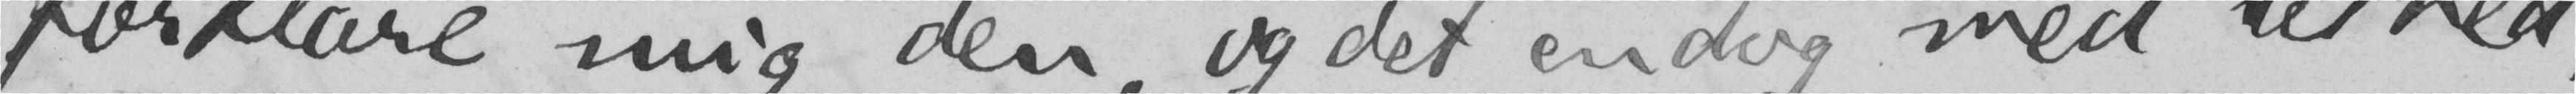forklare mig den, og det endog med lethed,
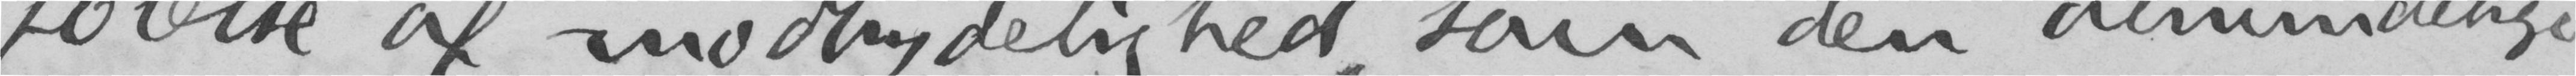følelse af modbydelighed, som den almindelige
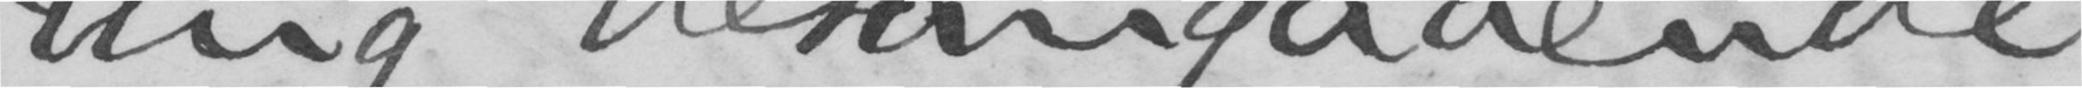ring desangaaende.
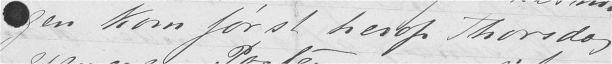gen kom først herop Thorsdag
